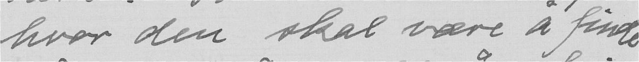hvor den skal være å finde
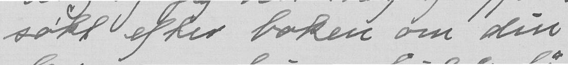søkt efter boken om din
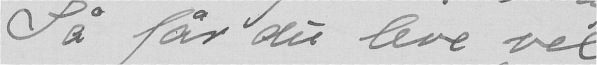Så får du leve vel
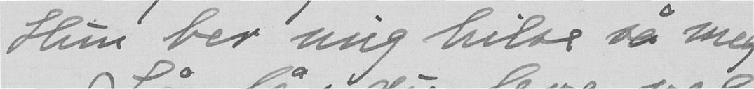Hun ber mig hilse så meget.
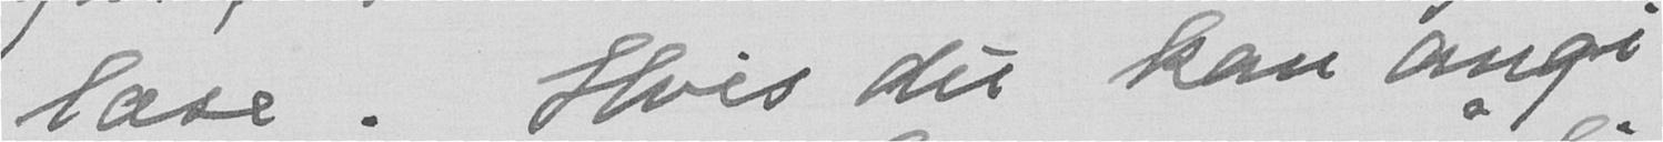læse. Hvis du kan angi
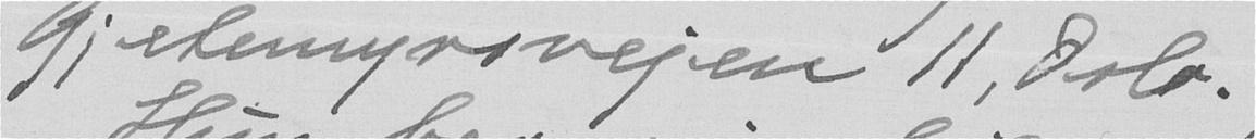Gjetemyrsvejen 11, Oslo.
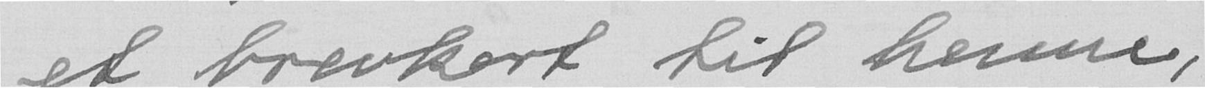et brevkort til henne,
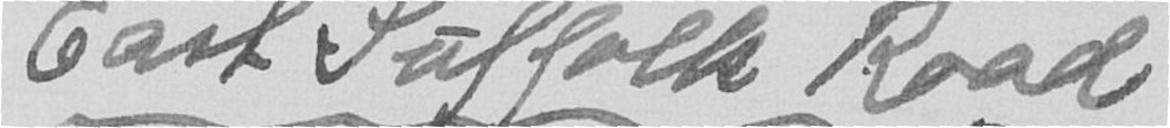East Suffolk Road
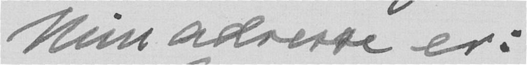Min adresse er:
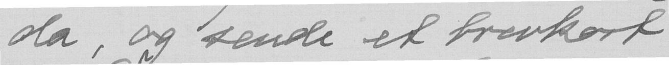da, og sende et brevkort
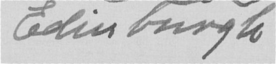Edinburgh
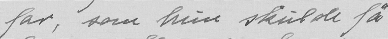far, som hun skulde få
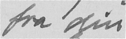fra din
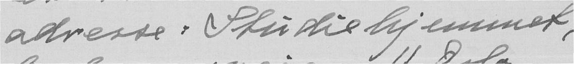adresse: Studiehjemmet,
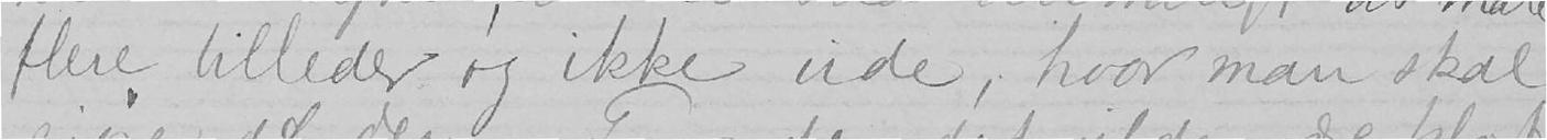flere billeder og ikke vide, hvor man skal
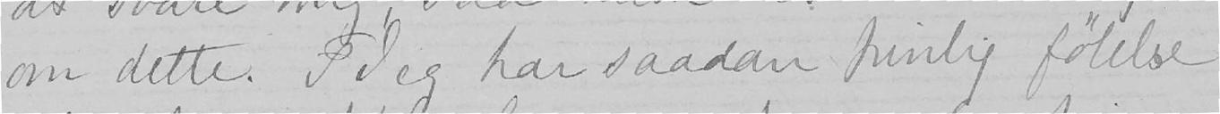om dette. T Jeg har saadan pinlig følelse
